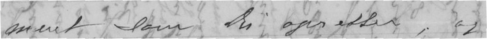ment som Du opretter, og
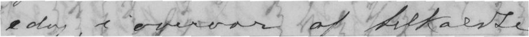eder, i overvær af tilkaldte
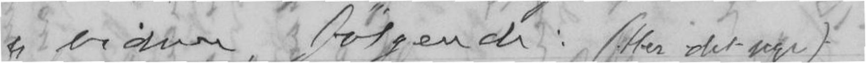vidner, følgende (Her det sige)
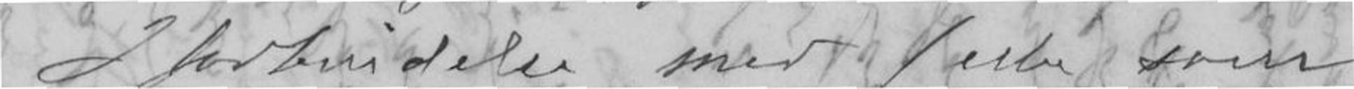I forbindelse med (eller som
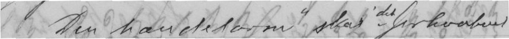Den handelorm skal det forhaabent-
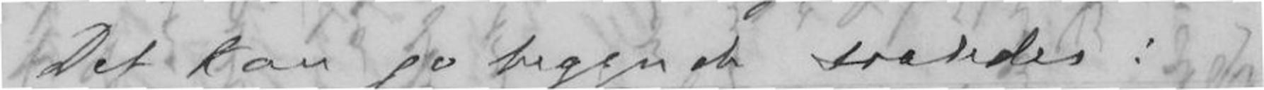Det kan jo begynde saaledes:
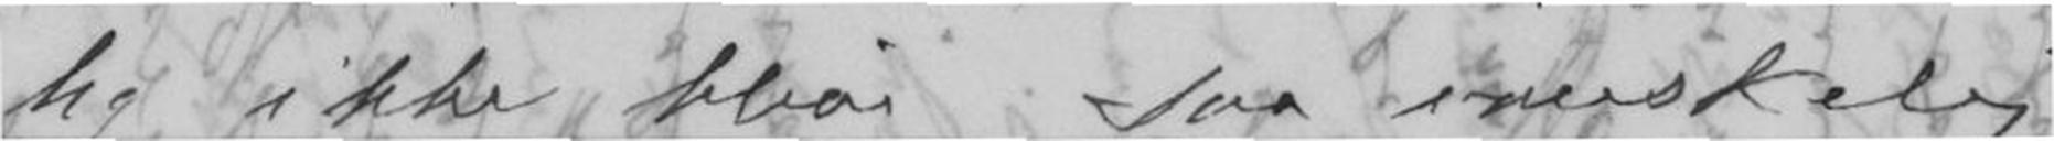lig ikke blive saa vanskelig
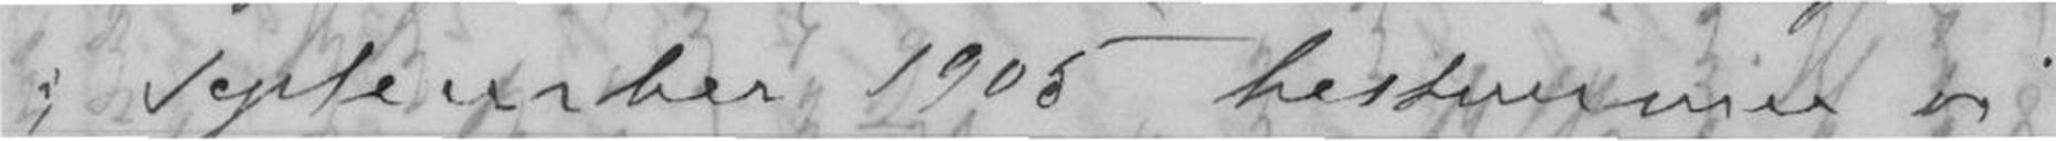september 1905 bestemmer vi
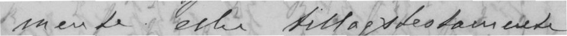mente eller tillægstestestamente
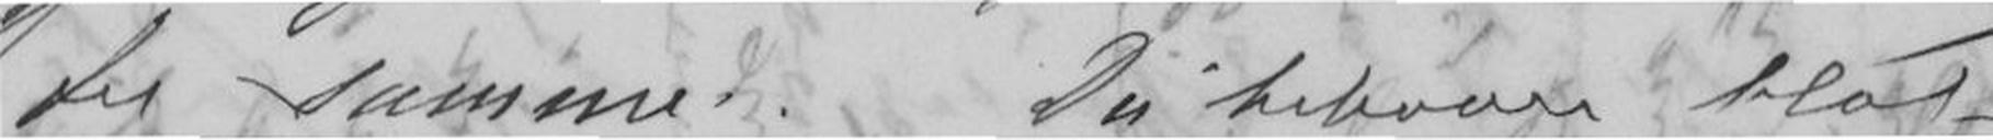til samme. Du behøver blot
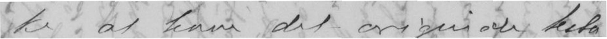ke at have det originale testa-
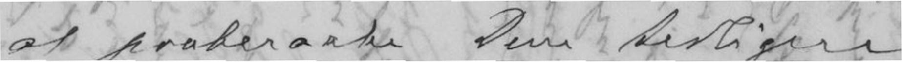at paaberaabe Dine tidligere
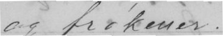og frøkener.
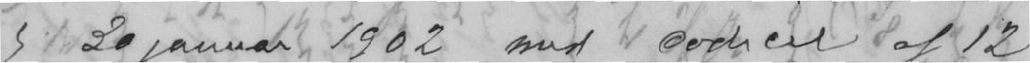20 januar 1902 med codicil af 12
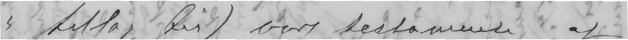tillæg til) vort testamente af
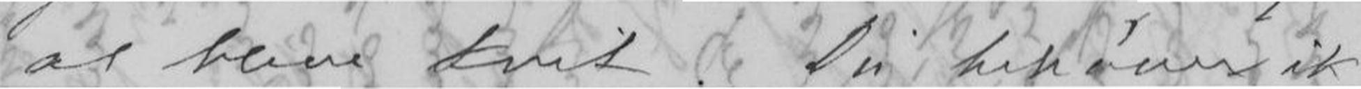at blive kvit. Du behøver ik-
